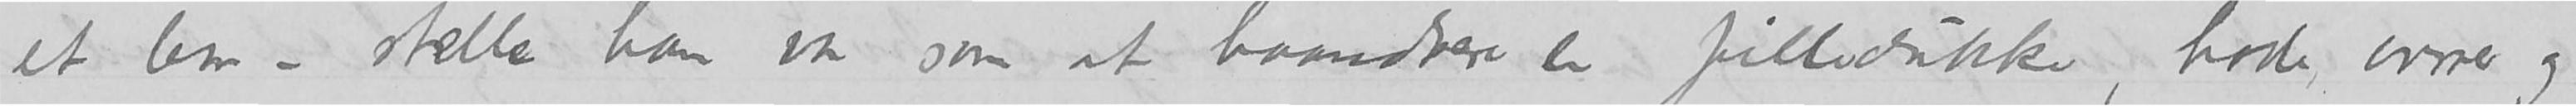et ben – stelle ham var som at haandtere en filledukke, hode, armer og
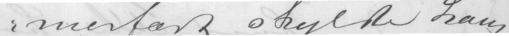merfæst skyldte hans
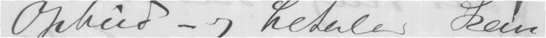Opbud - og betaler kan
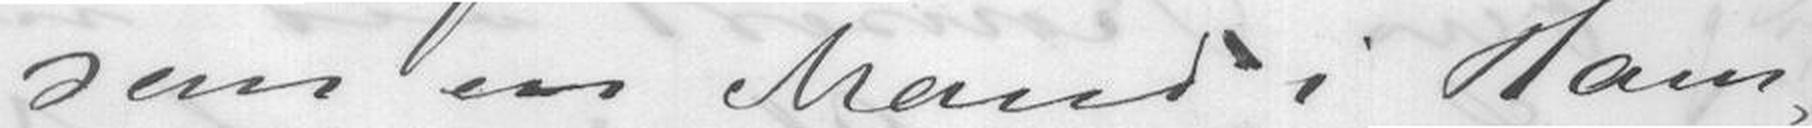som en Mand i Ham
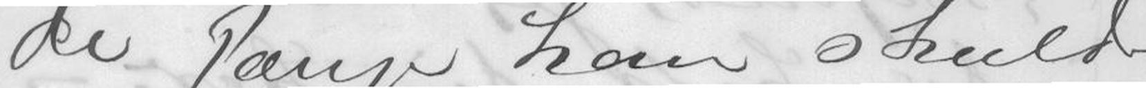de Pænge han skulde
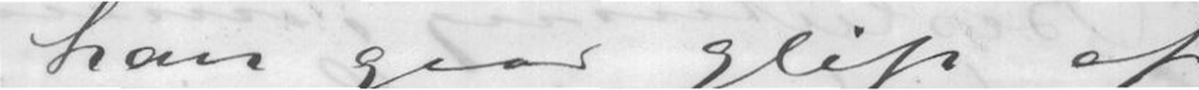han gaar glip af
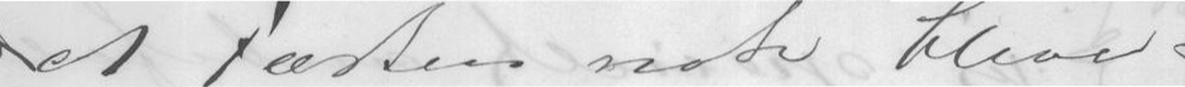at Fæsten nok blive
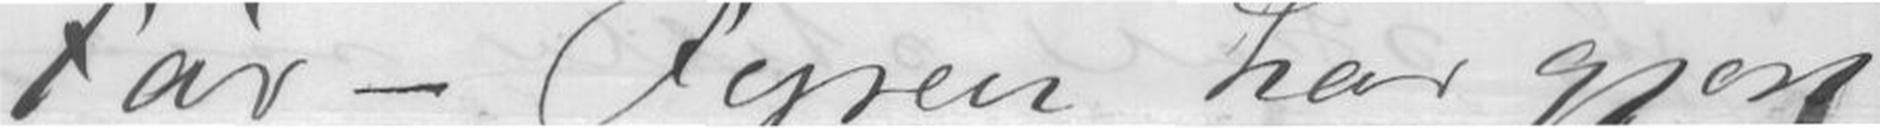Far - Fyren har gjort
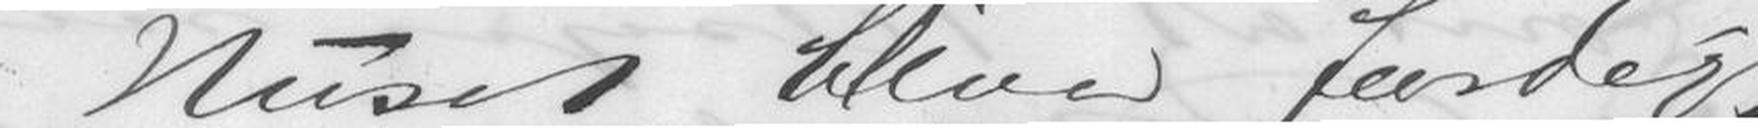Huset bliver færdigt
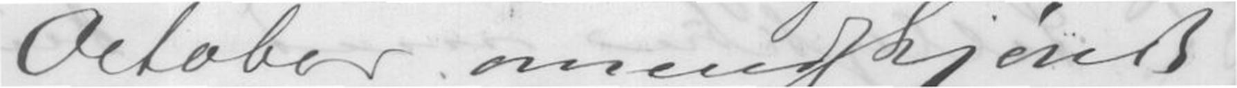October omendskjøndt
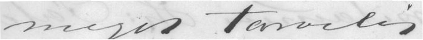meget trvelig
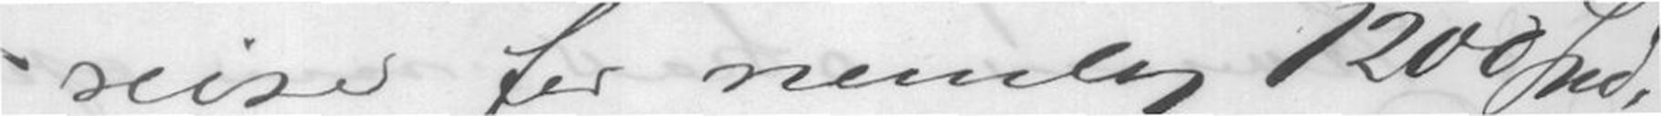reise for nemlig 1200 Spd,
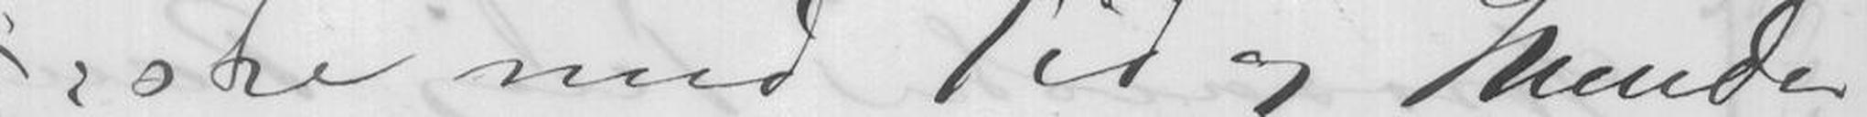ske med Tid og Stunder
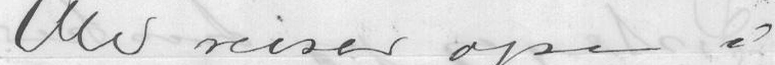Ole reiser ogsaa i
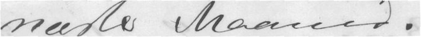næste Maaned.
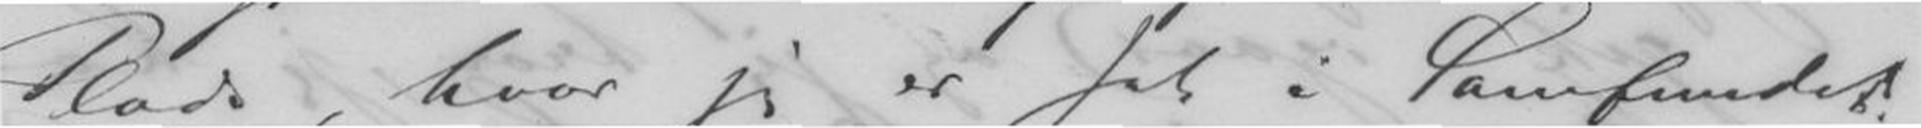Plads, hvor jeg er sat i Samfundet.
